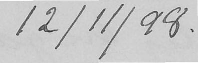12/11/98.
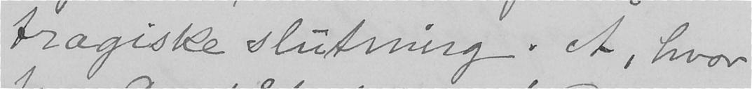tragiske slutning. Å, hvor
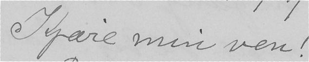Kjære min ven!
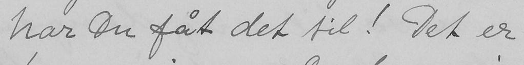har Du fåt det til! Det er
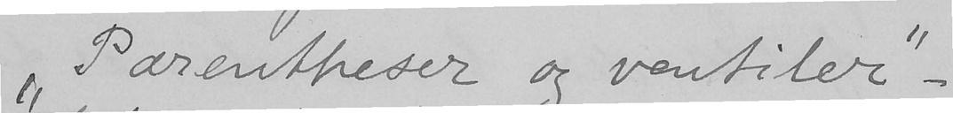"Parentheser og ventiler" -
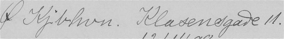Ø Kjbhvn. Klasensgade 11.
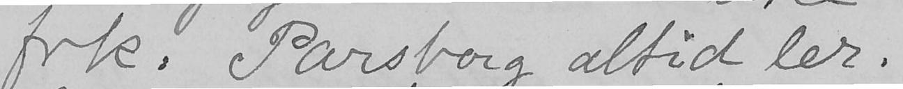frk. Parsberg altid ler.
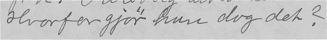Hvorfor gjør hun dog det?
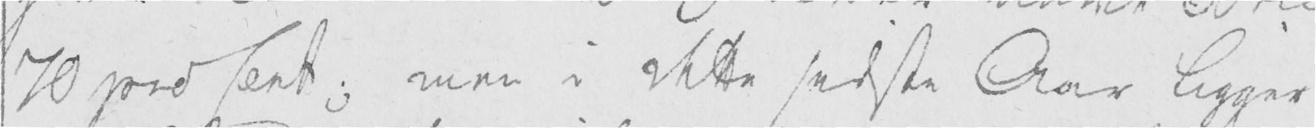70 prosent; men i dette sidste Aar ligger
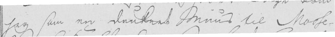jeg som en druknet Muus til Mosse-
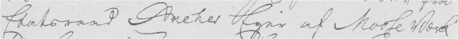Etatsraad Ancher Eyer af Mosse Værk
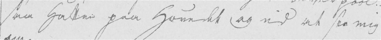saa Hatten paa Hovedet og ud at see mig
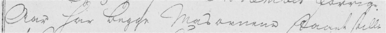Aar har begge Masovnene staaet stille
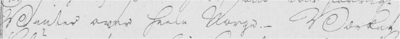Vinter over heele Norge. - Værket
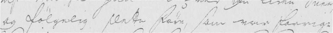og følgelig slette Føre som var forrige
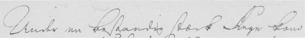Under en bestandig stærk Regn kom
